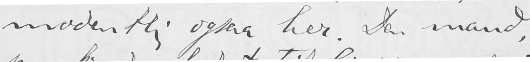modentlig ogsaa her. Den mand,
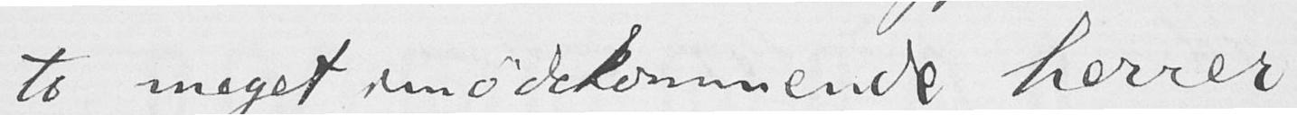to meget imødekommende herrer
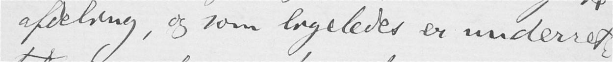afdeling, og som ligeledes er underret-
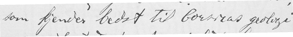som kjender bedst til Corsicas geologi
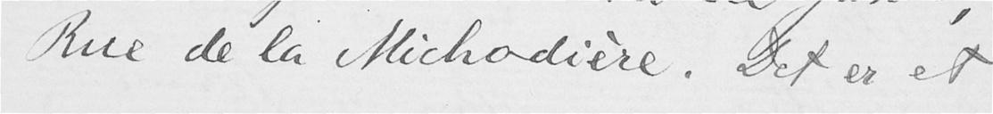Rue de la Michodière. Det er et
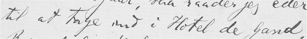til at tage ind i Hotel de Gand,
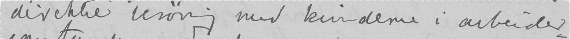direkte berøring med kvinderne i arbeider-
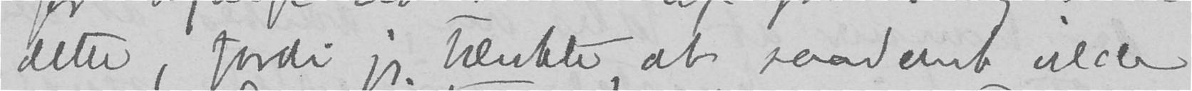dette, fordi jeg tænkte, at saadant vilde
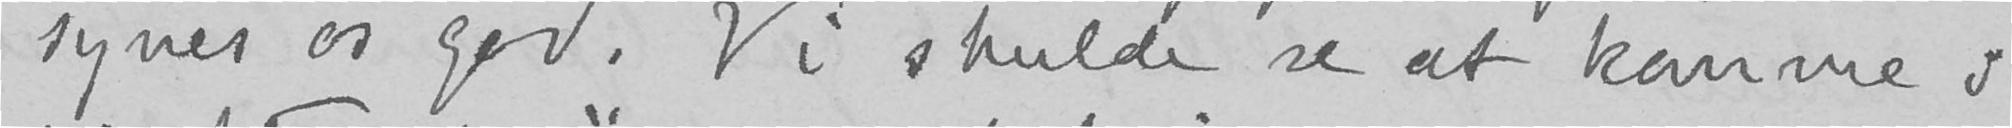synes os god. Vi skulde se at komme i
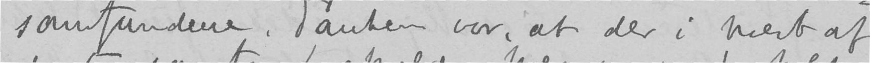samfundene. Tanken var, at der i hvert af
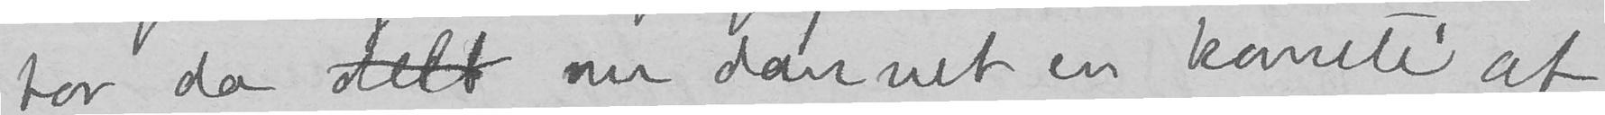har da delt nu dannet en komité af
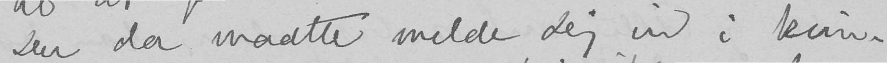Du da maatte melde Dig ind i kvin-
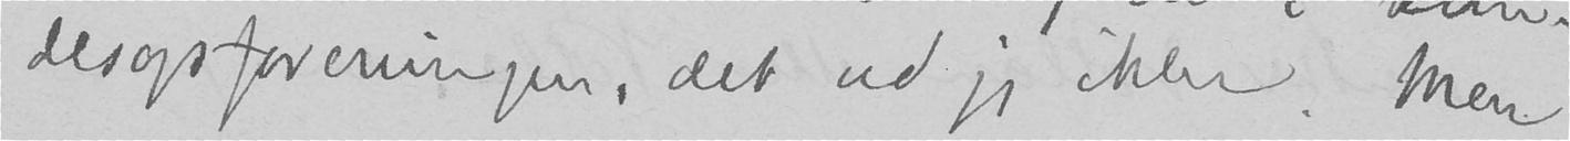desagsforeningen, det ved jeg ikke. Men
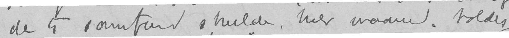de 5 samfund skulde, hver maaned, holdes
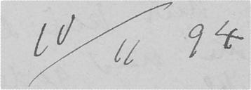11/11 94
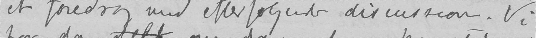et foredrag med efterfølgende discussion. Vi
